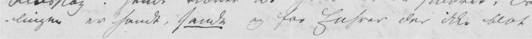Camilla Collett.
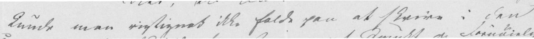man rigtignok ikke falde paa at
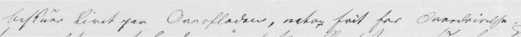punctum quæstionis
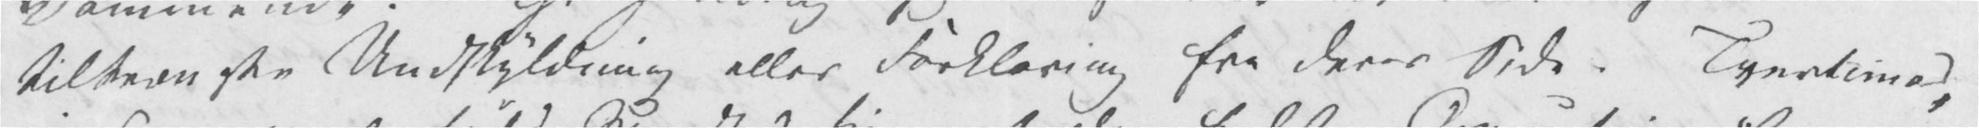tiltrængte Undskyldning eller Forklaring fra Deres Side. Tvertimod,
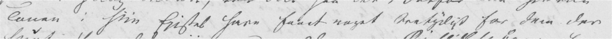Tonen i hiin Epistel have faaet noget tvetydigt for dem der
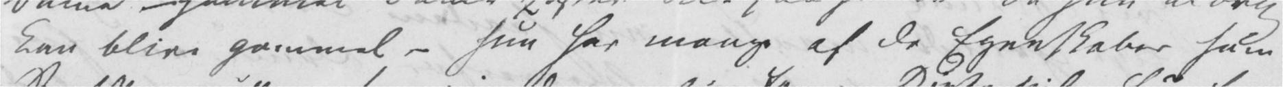almindelige løgnagtige Taareperser, saa læste man den nok ud, naar man forresten holdt ud at læse den.
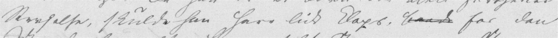Revselse, skulde han have lidt Klaps, baade for den
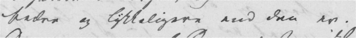og dog indeholdt de intet ualmindelig Sørgeligt, ingen Rædsler, intet af alt det der findes
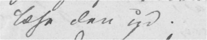Jeg tilskrev det den Skræk alle Ældre
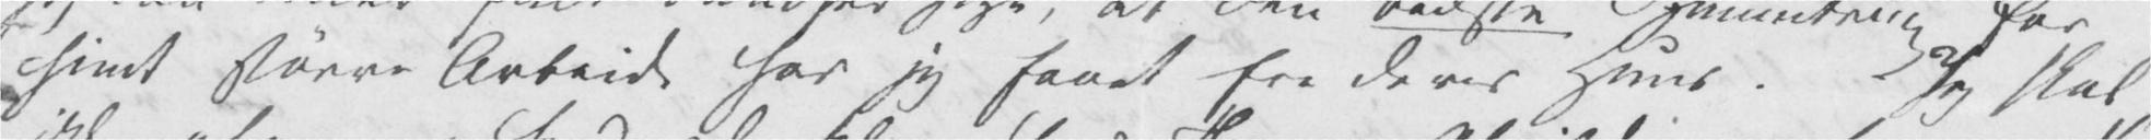hiint større Arbeide har jeg faaet fra Deres Huus. Jeg skal
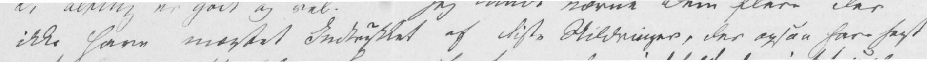glæde os over, og som vil
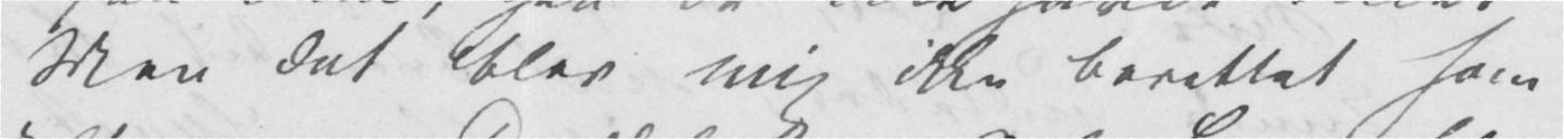som om De havde udtalt nogen Daddel over Arbe Bogen selv, og heller ikke beholdt jeg Indtrykket deraf.
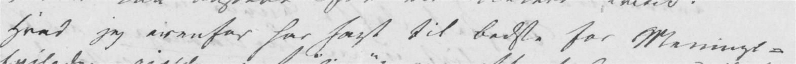Hvad jeg ovenfor har sagt til bedste for Menings-
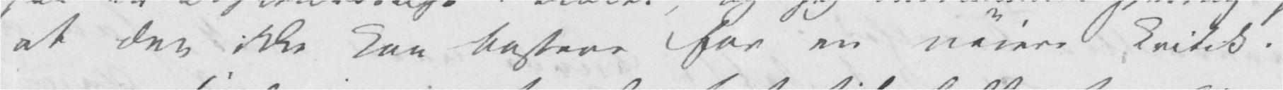at den ikke kan bestaae for en nøiere Kritik.
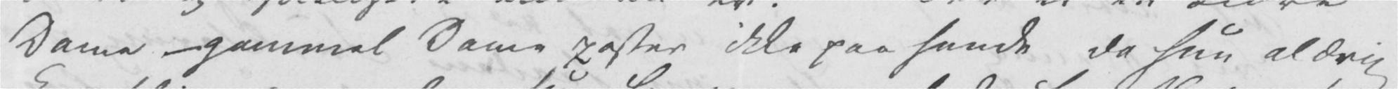1000 Gange Dynget sammen i andre Romaner. Det maae altsaa være Sandheden i denne der griber, thi var det en af disse
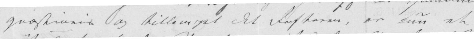og tillempet det Frfteren,
